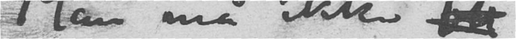Man må ikke bli
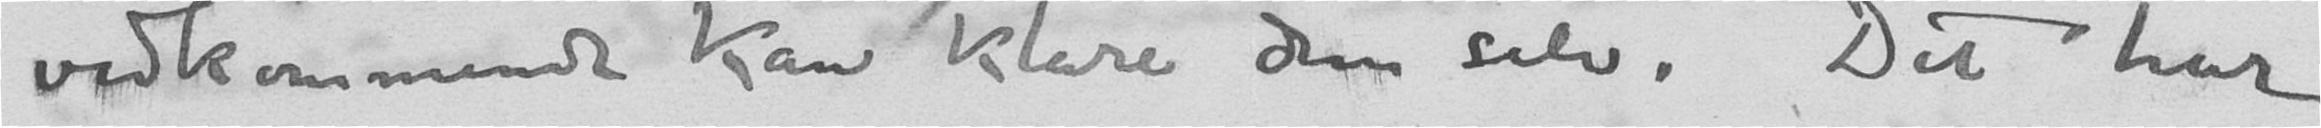vedkommende kan klare dem selv. Det har
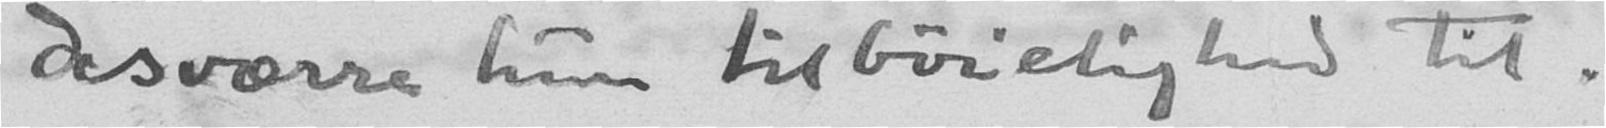desværre hun tilbøielighed til.
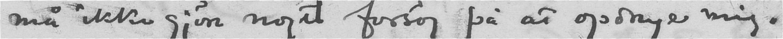må ikke gjøre noget forsøg på at opdrage mig.
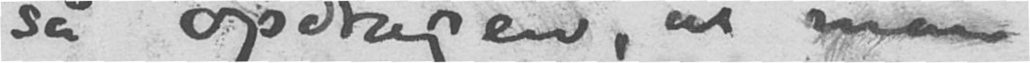så opdragen, at man
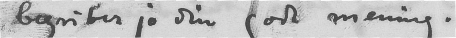begriber jo din gode mening.
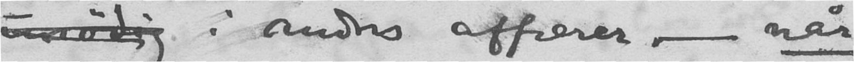unødig i andres affærer - når
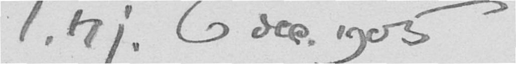T.hj. 6 dec. 1905
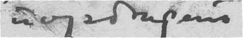uopdragent
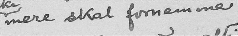mere skal fornemme
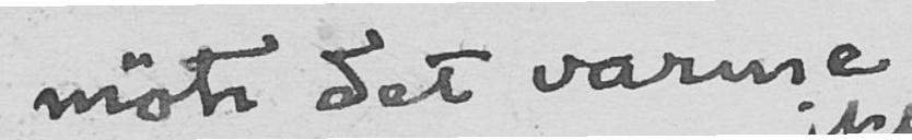møte det varme
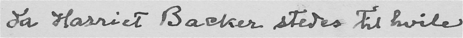da Harriet Backer stedes til hvile
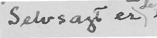Selvsagt er
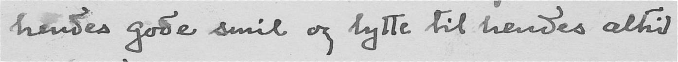hendes gode smil og lytte til hendes altid
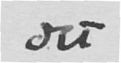det
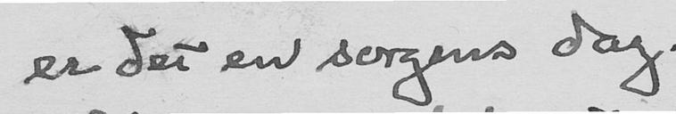er det en sorgens dag.
